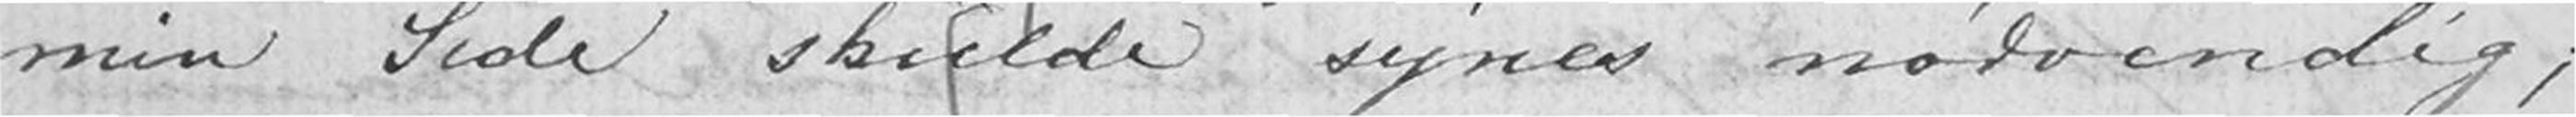min Side skulde synes nødvendig;
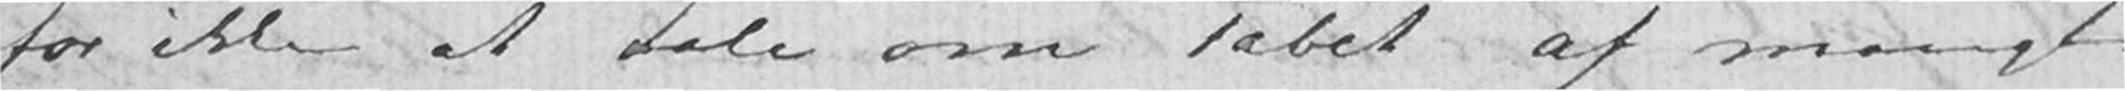for ikke at tale om Tabet af mangt
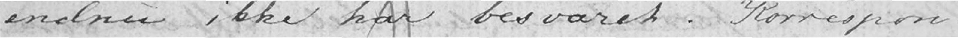endnu ikke har besvaret. Korrespon-
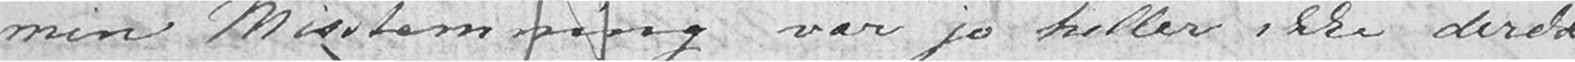min Misstemning var jo heller ikke direkte
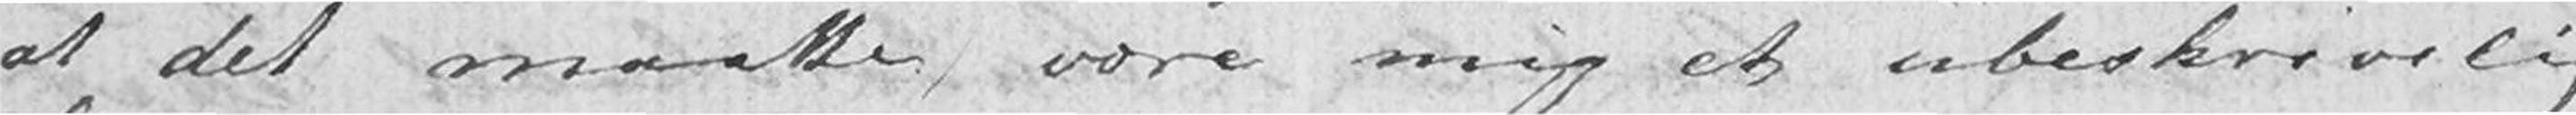at det maatte være mig et ubeskrivelig
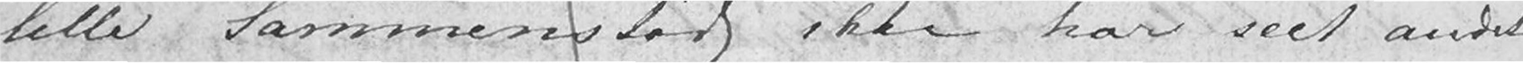lille Sammenstød ikke har seet andet
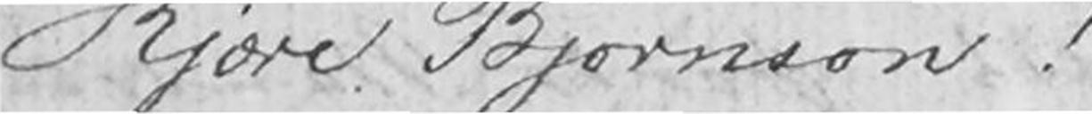Kjære Bjørnson!
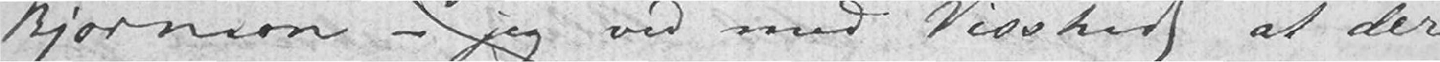Bjørnson - jeg ved med Visshed at der
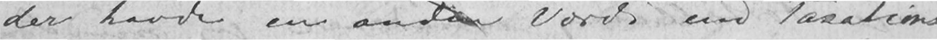der havde en anden Værdi end Taxations-
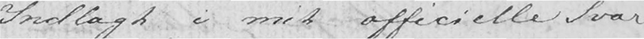Indlagt i mit officielle Svar
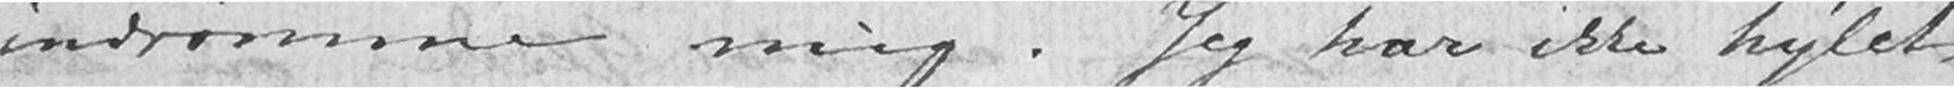indrømme mig. Jeg har ikke hylet
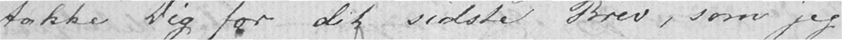takke Dig for dit sidste Brev, som jeg
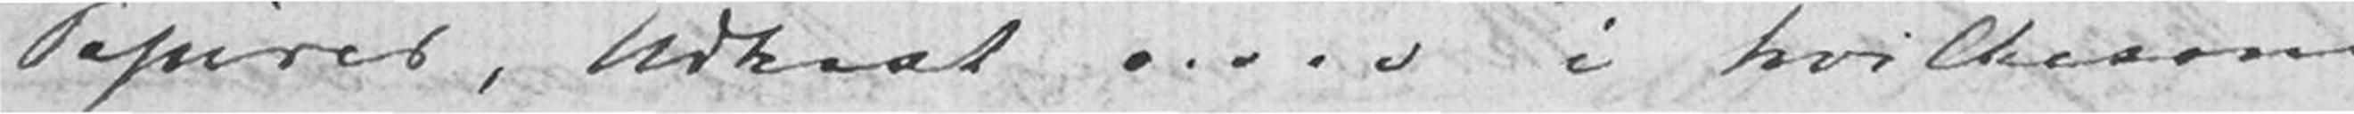Papirer, Udkast o.s.v i hvilkesom-
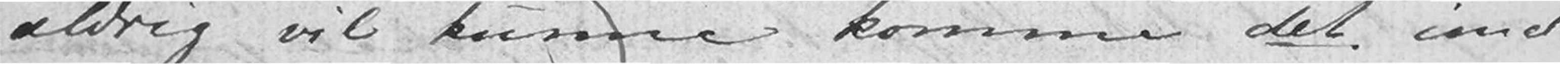aldrig vil kunne komme det imel-
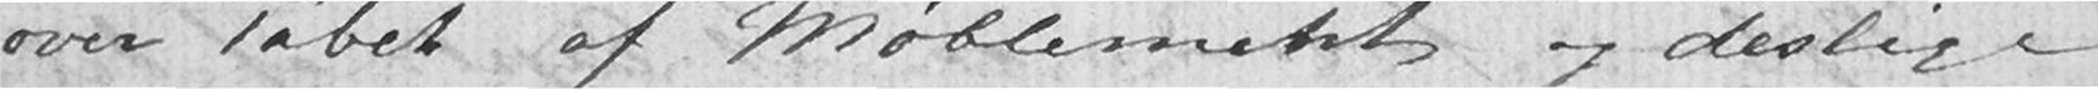over Tabet af Møblement og deslige,
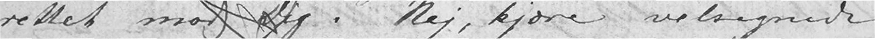rettet mod Dig. Nej, kjære velsignede
